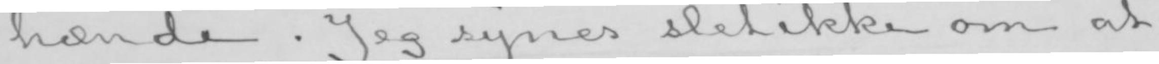hænde. Jeg synes sletikke om at
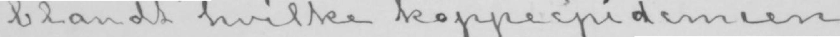blandt hvilke koppeepidemien
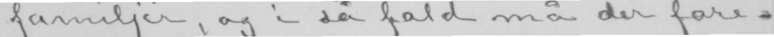familjer, og i så fald må der fore-
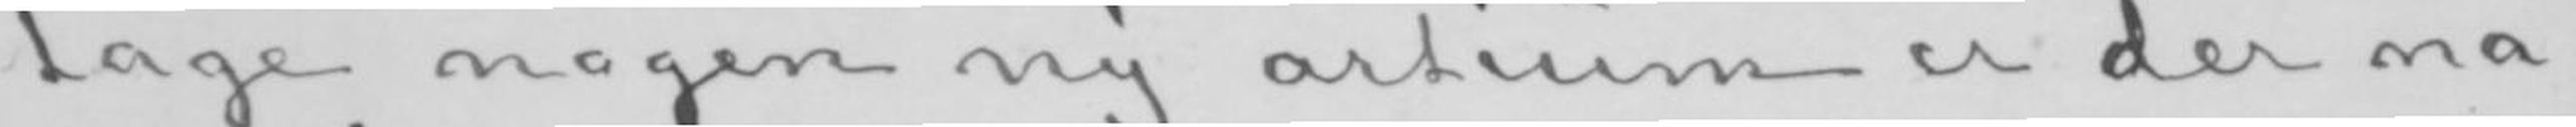tage nogen ny artium er der na-
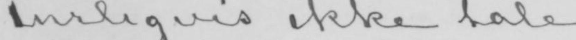turligvis ikke tale.
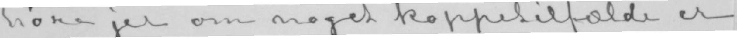høre jer om noget koppetilfælde er
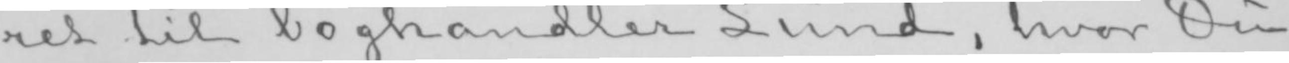ret til boghandler Lund, hvor Du
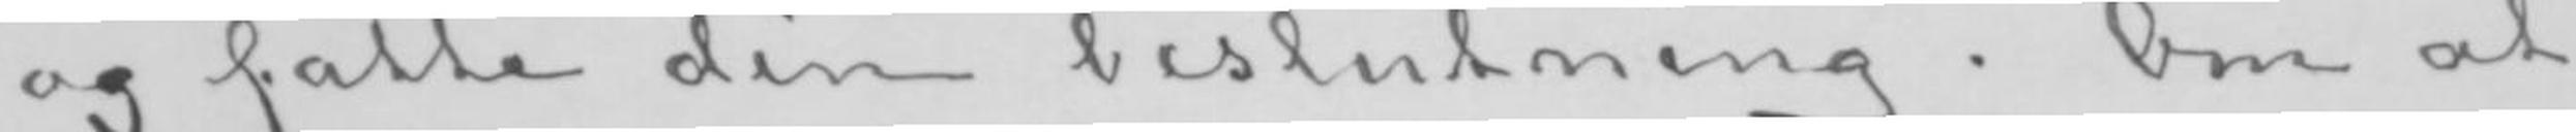og fatte din beslutning. Om at
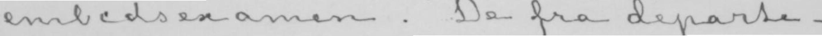embedsexamen. De fra departe-
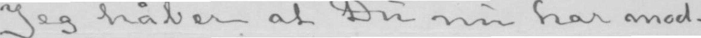Jeg håber at Du nu har mod-
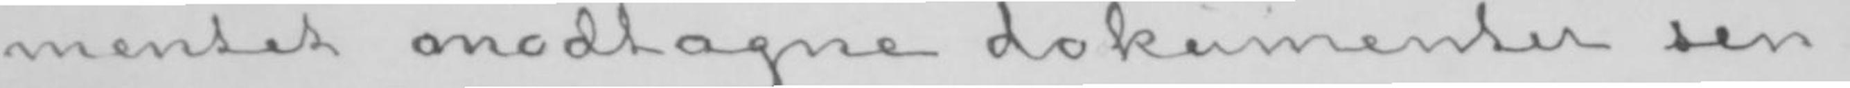mentet omodtagne dokumenter sen-
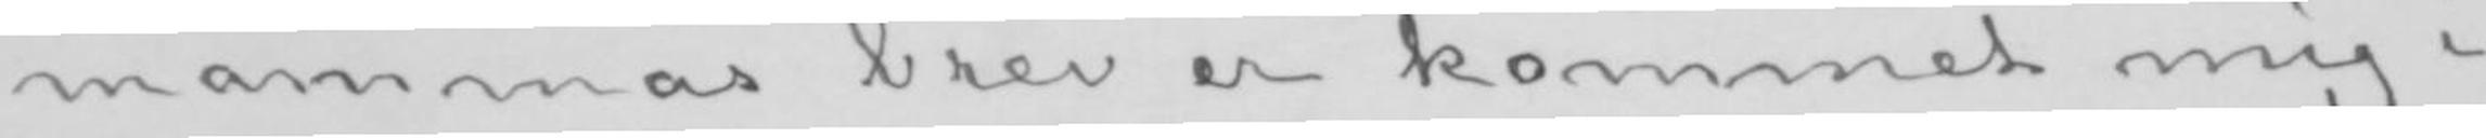mammas brev er kommet mig i
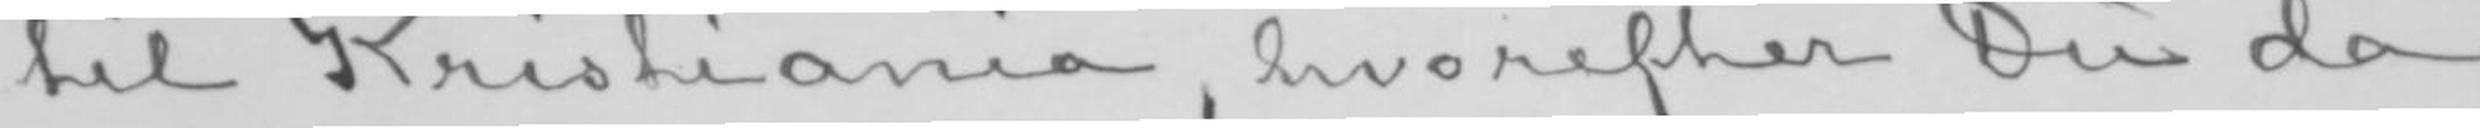til Kristiania, hvorefter Du da
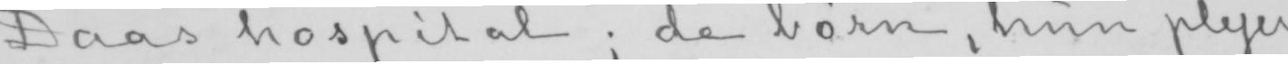Daas hospital; de børn, hun plejer
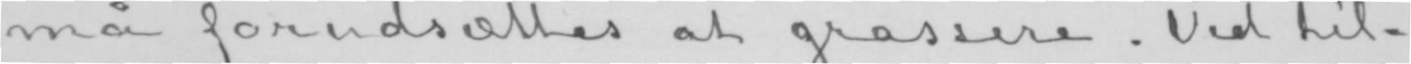må forudsættes at grassere. Ved til-
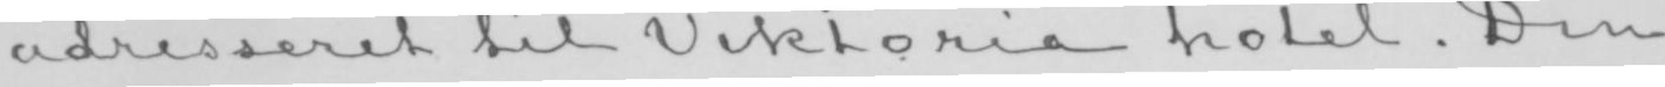adresseret til Viktoria hotel. Din
